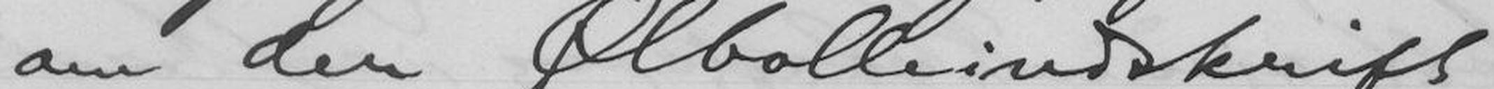om den Ølbolleindskrift,
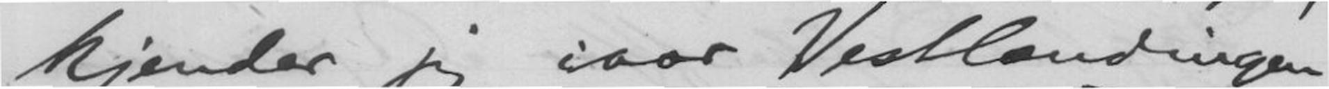kjender jeg iaar Vestlændingen
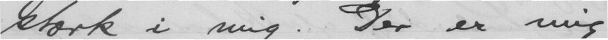stærk i mig. Der er mig
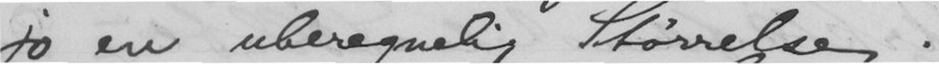jo en uberegnelig Størrelse.
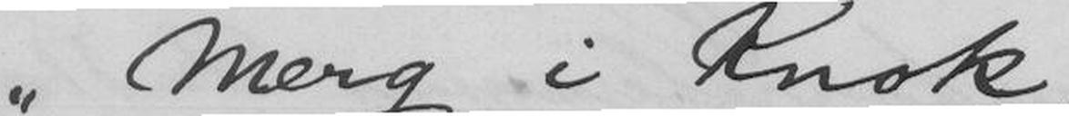" Merg i Knok,
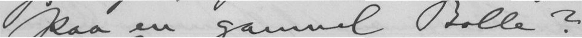paa en gammel Bolle?
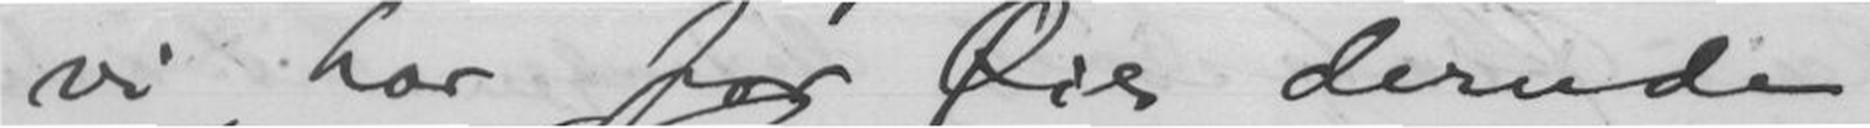vi har for Øie derude
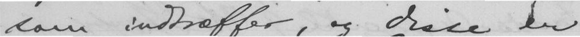som indtræffer, og disse er
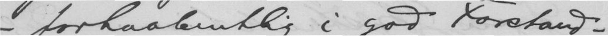- forhaabentlig i god Forstand -
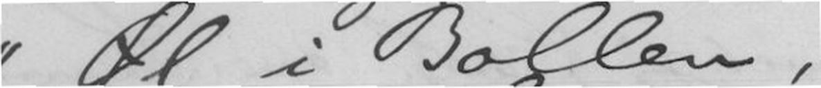" Øl i Bollen,
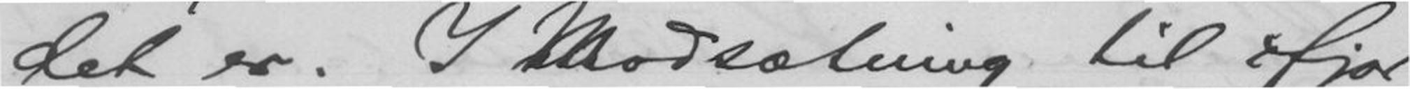det er. I Modsætning til ifjor,
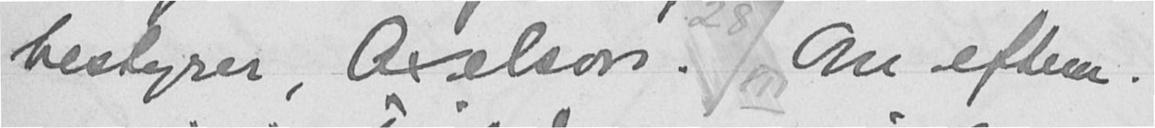bestyrer, Axelson. Om efterm.
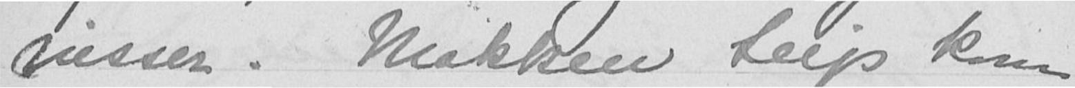nisser. Makken Seip kom
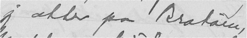jeg atter paa Bratøen,
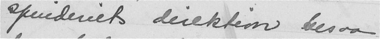spinderiets direktion besaa
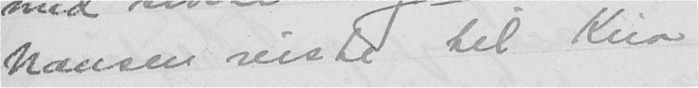Nansen reiste til Kria
